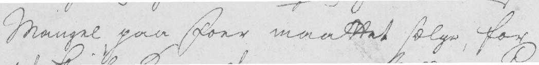Mangel paa Foer maattet sælge, for
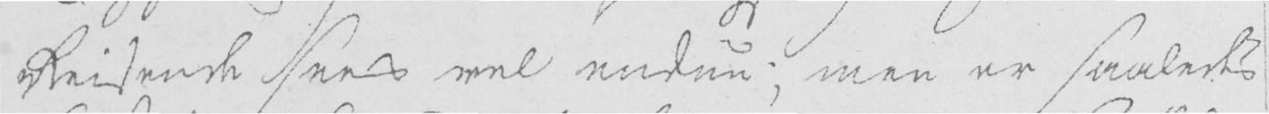Reisende sees vel endnu; men er saaledes
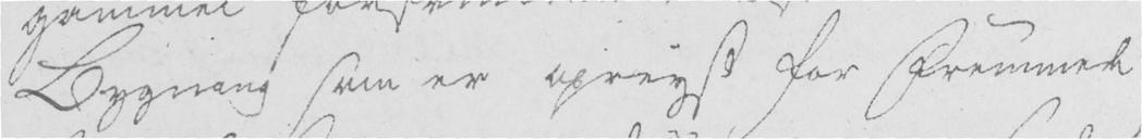Bygning som er opreyst for Fremmed
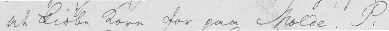at kiøbe Korn for paa Molde. P.
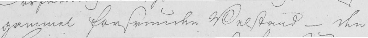gammel forsvunden Velstand - den
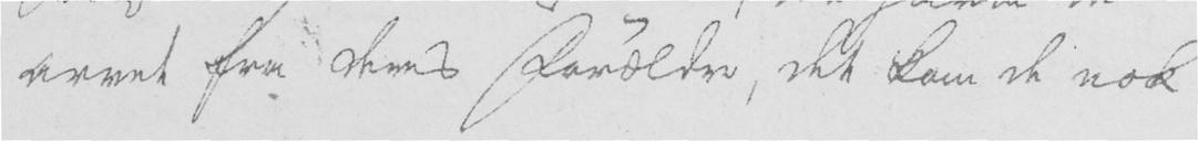arvet fra deres Forældre, det kom de nok
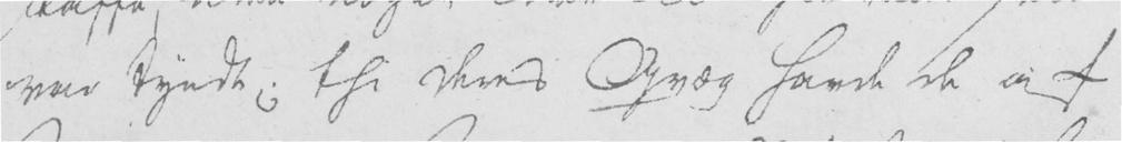var tyndt; thi deres Qvæg havde de af
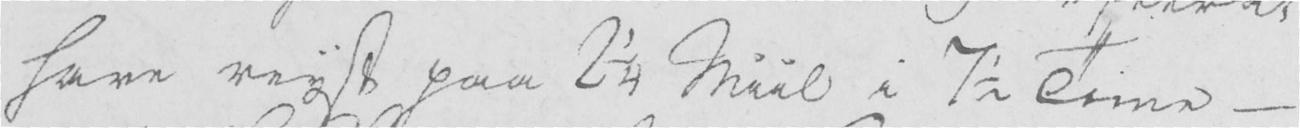have reyst paa 2 1/4 Miil i 7 1/2 Time -
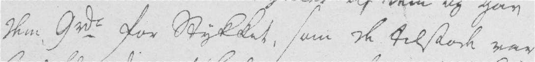dem 9 rde for Stykket, som de tilstode var
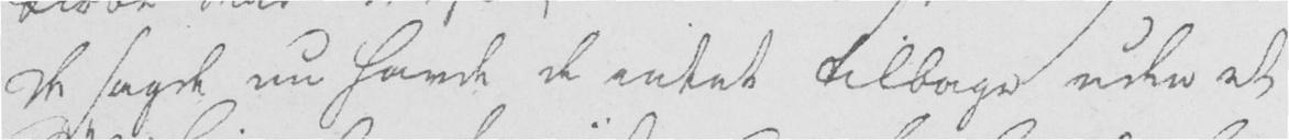de sagde nu havde de intet tilbage uden et
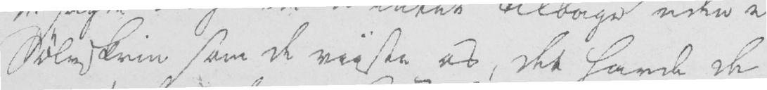Sølvskrin som de viiste os, det havde de
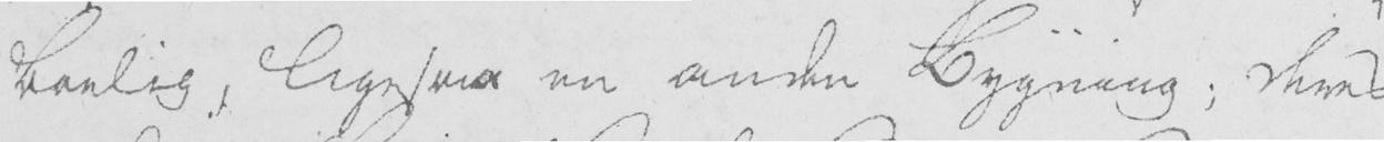boelig, ligesom en anden Bygning; deres
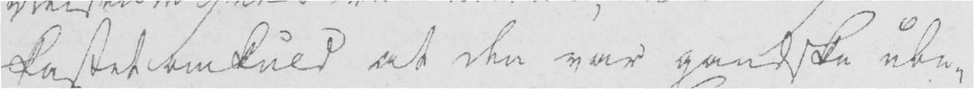kastet omkuld at den var gandske ube-
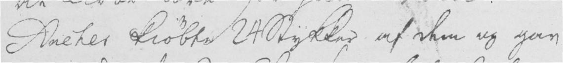Ancher kiøbte 24 Stykker af dem og gav
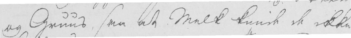og Gruus, saa at Melk kunde de ikke
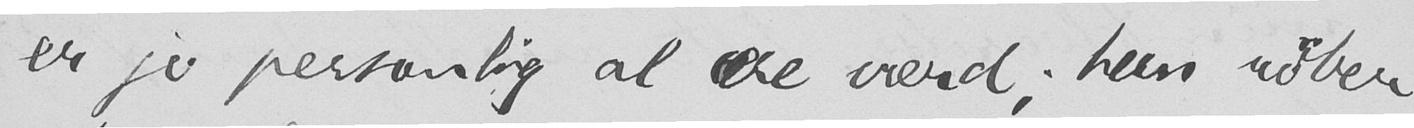er jo personlig al ære værd; hun røber
